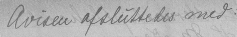Avisen afsluttedes med:
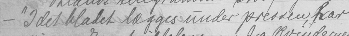- "Idet bladet lægges under pressen, faar
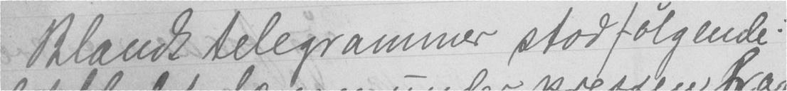Blandt telegrammer stod følgende:
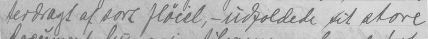terdragt af sort fløiel, - udfoldede sit store
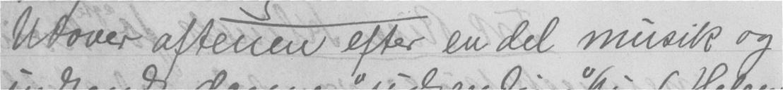Udover aftenen efter en del musik og
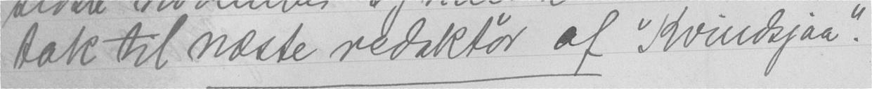tak til næste redaktør af "Kvindsjaa".
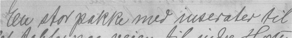En stor pakke med inserater til
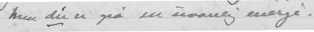Men du er også en uvanlig energi.
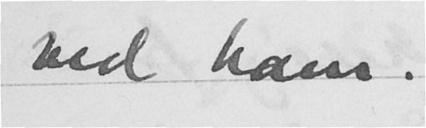ved ham.
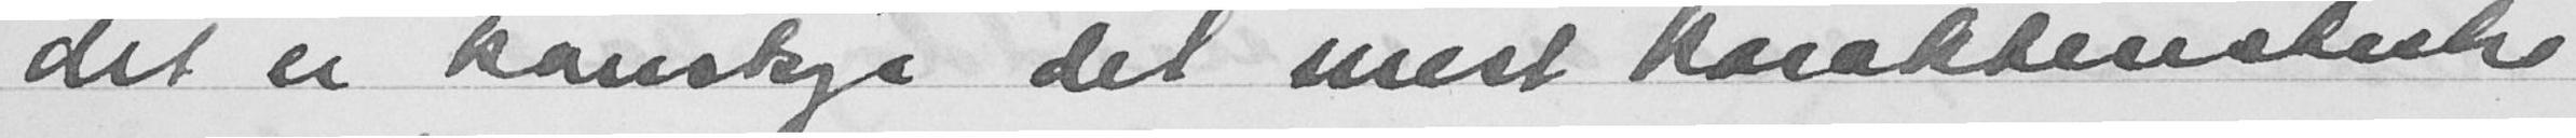det er kanskje det mest karekteristiske
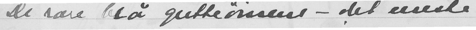De rare blå gutteøinenen - det eneste
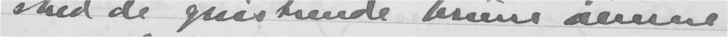med de gnistrende brune øinene
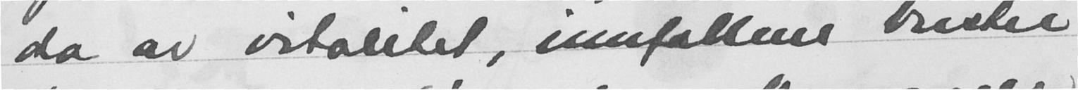da av vitalitet, infallene brister
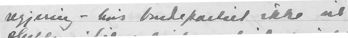regjering - hvis bondepartiet ikke vil
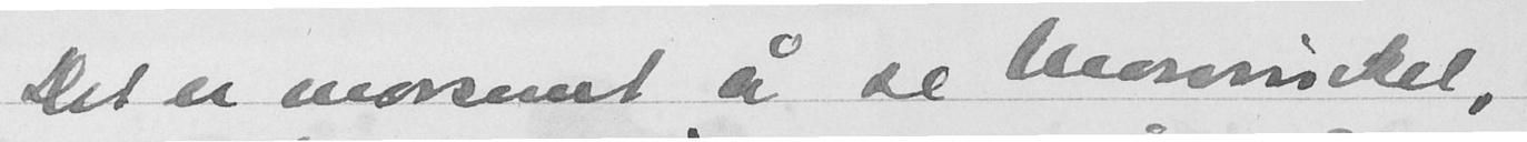Det er morsomt å se Mowinckel,
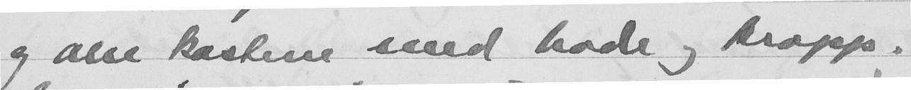og alle kastene med hode og kropp,
